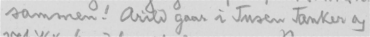sammen! Arild gaar i Tusen Tanker og
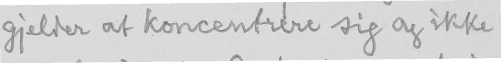gjelder at koncentrere sig og ikke
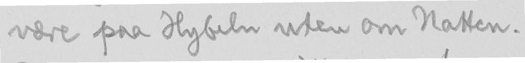være paa Hybeln uten om Natten.
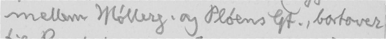mellem Møllerg. og Pløens Gt., bortover
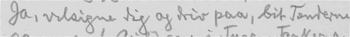Ja, velsigne dig og driv paa, bit Tænderne
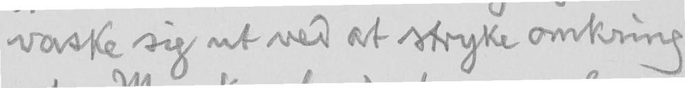vaske sig ut ved at stryke omkring
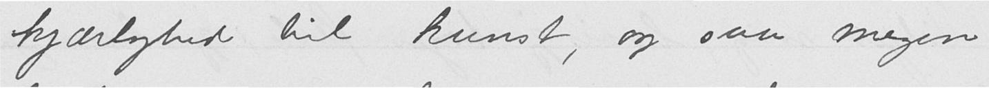kjærlighed til kunst, og saa megen
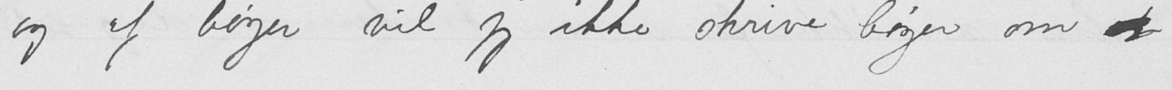og af bøger vil jeg ikke skrive bøger om e
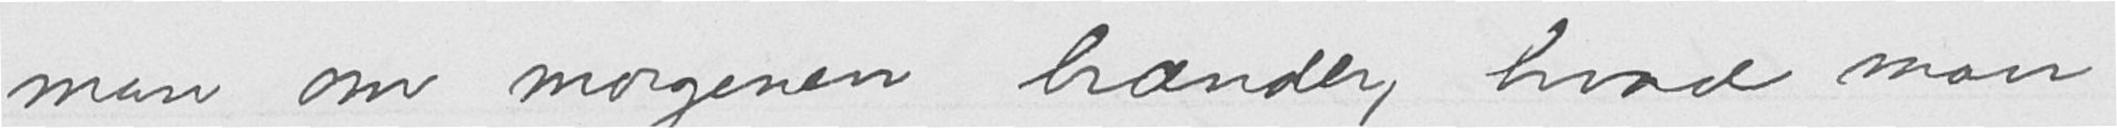man om morgenen brænder, hvad man
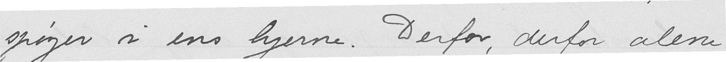spøger i ens hjerte. Derfor, derfor alene
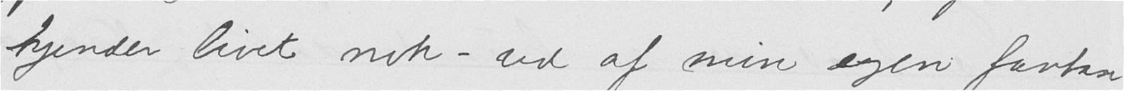kjender livet nok - ud af min egen forstand
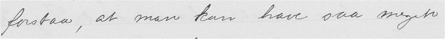forstaa, at man kan have saa meget
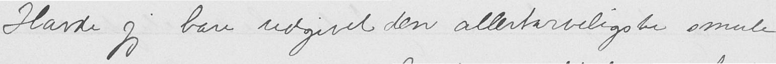Havde jeg bare udgivet den allertarveligste smule
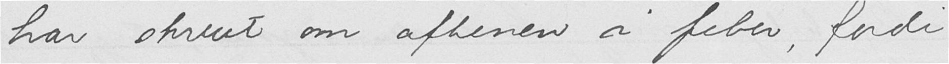har skrevet om aftenen i feber, fordi
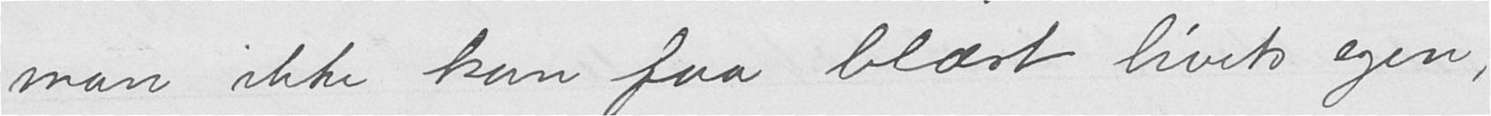man ikke kan faa blæst livets egen
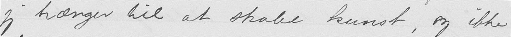jeg trænger til at skabe kunst, og ikke
